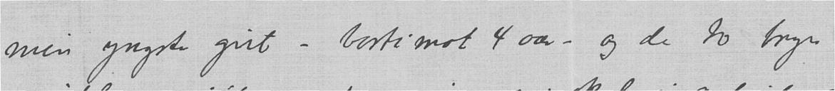min yngste gut – bortimot 4 aar - og de to bryr
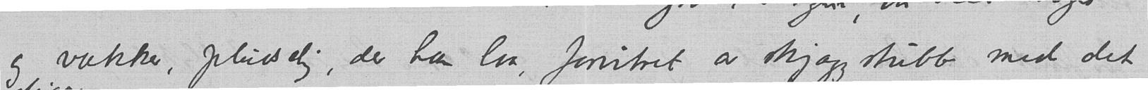og vakker, pludselig, der han laa, forvitret av skjæggstubb med det
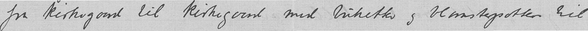fra kirkegaard til kirkegaard med buketter og blomsterpotter til
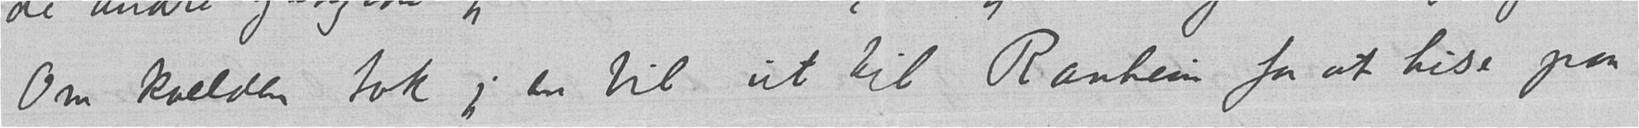Om kvelden tok jeg en bil ut til Ranheim for at hilse paa
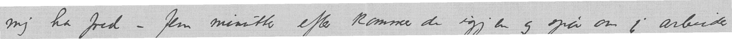mig ha fred – fem minutter efter kommer de igjen og spør om jeg arbeider
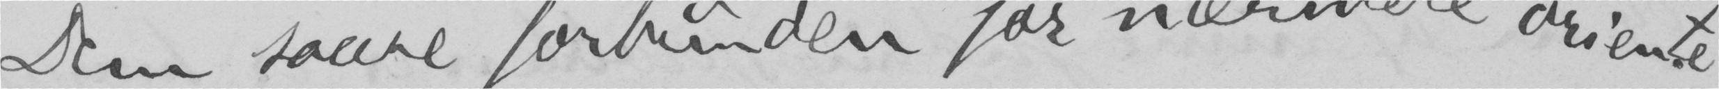Dem saare forbunden for nærmere oriente-
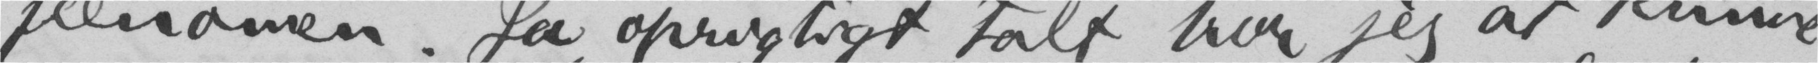fænomen. Ja, oprigtigt talt, tror jeg at kunne
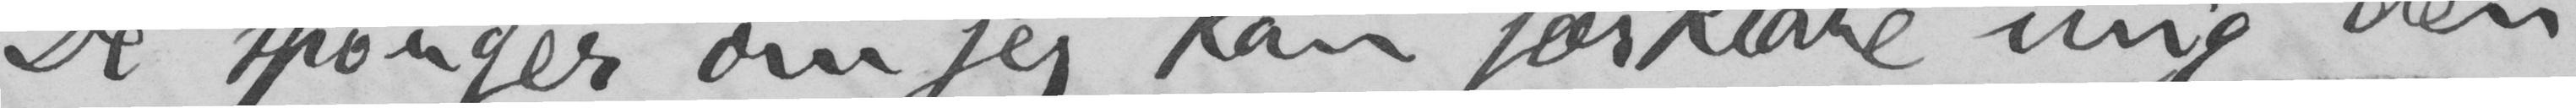De spørger, om jeg kan forklare mig den
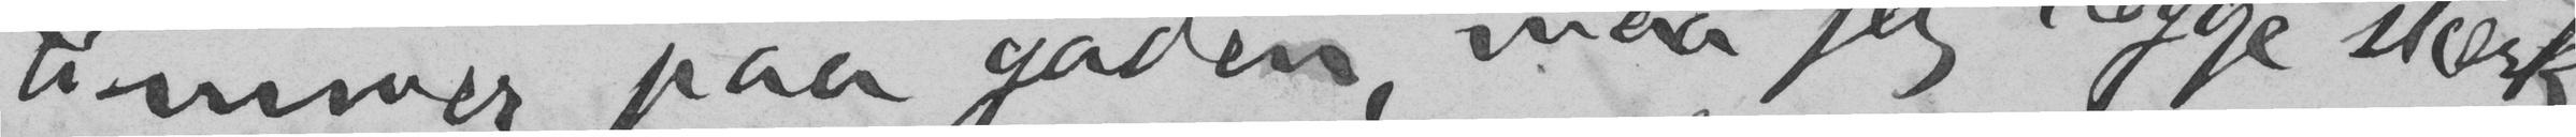timmer paa gaden, maa jeg lægge stærk
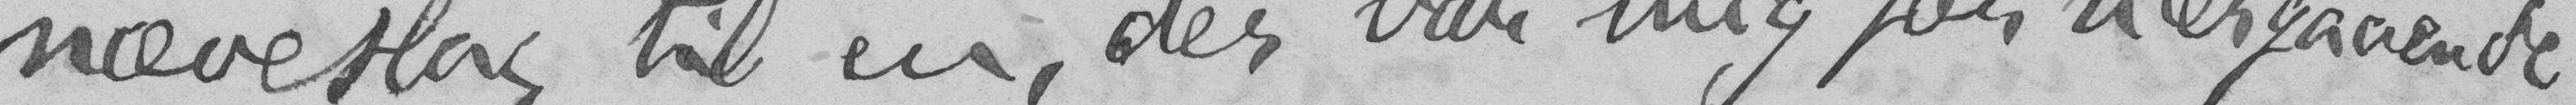næveslag til en, der var mig for nærgaaende)
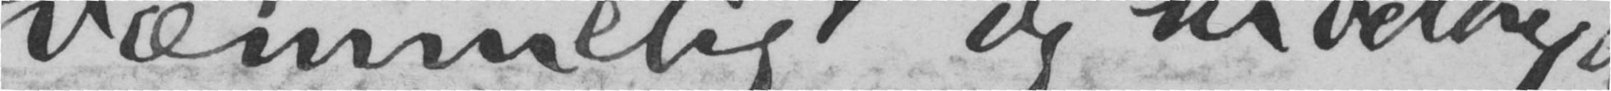væmmeligt og modbyde-
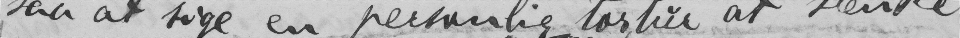saa at sige en personlig tortur at Tænke
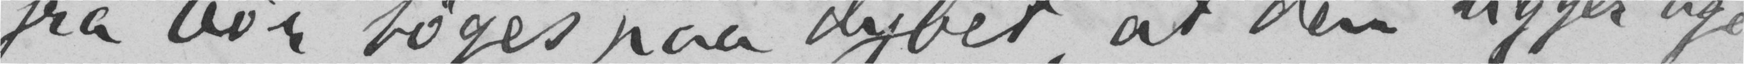fra bør søges paa dybet, at den ligger lige
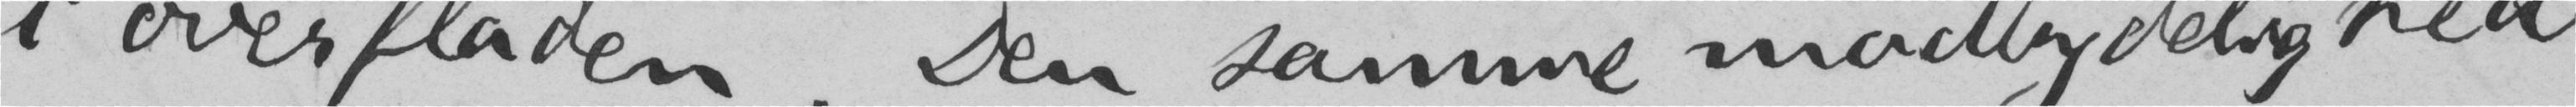i overfladen. Den samme modbydelighed
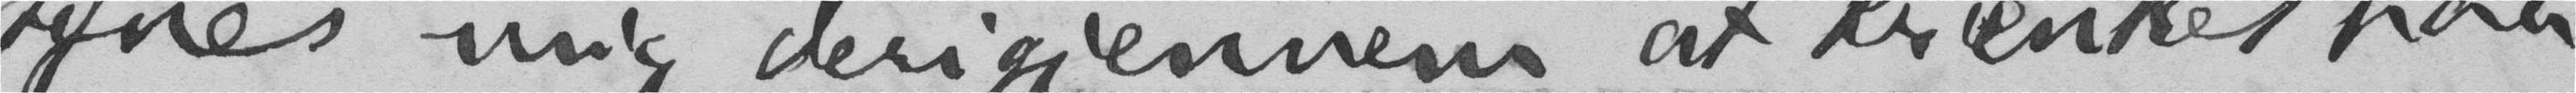synes mig derigjennem at krænkes paa
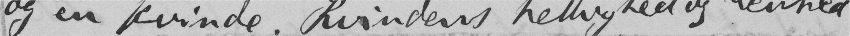og en kvinde. Kvindens hellighed og renhed
